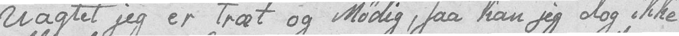Uagtet jeg er træt og Mødig, saa kan jeg dog ikke
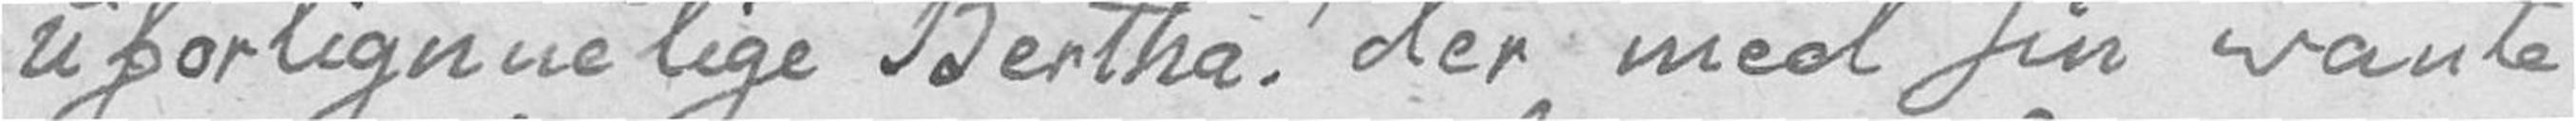uforlignnelige Bertha! der med sin vante
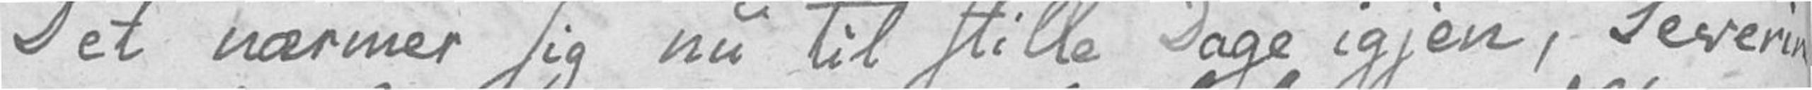Det nærmer sig nu til stille Dage igjen, Severin
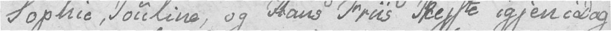Sophie, Pouline, og Hans Friis Rejste igjen i Dag
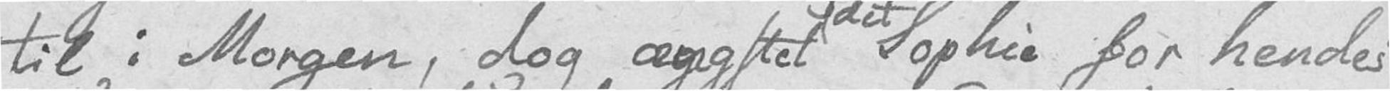til i Morgen, dog ængstet det Sophie for hendes
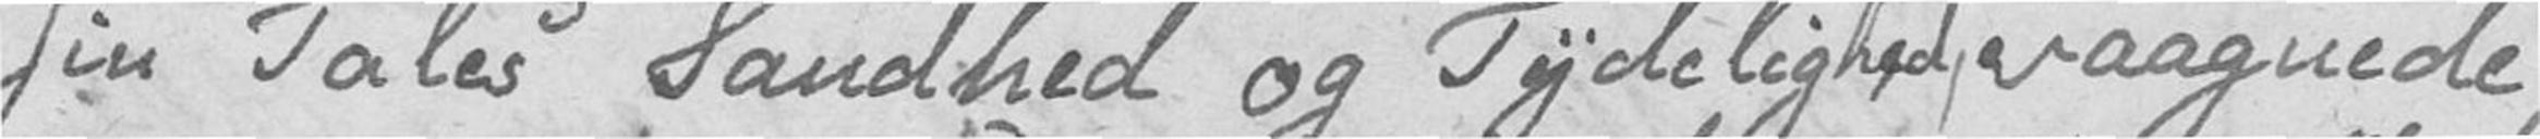sin Tales Sandhed og Tydelighed vaagnede
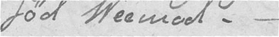sød Veemod. –
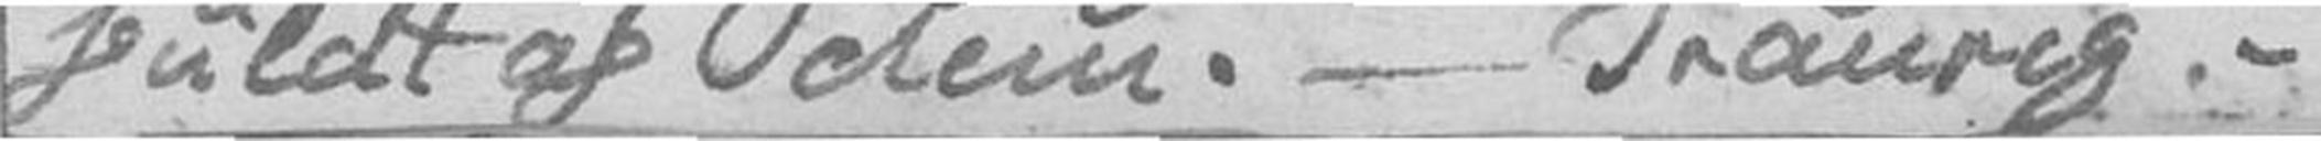fuldt af dem. – Traurig. –
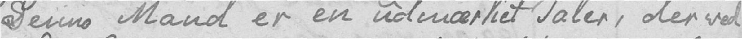Denne Mand er en udmærket Taler, der ved
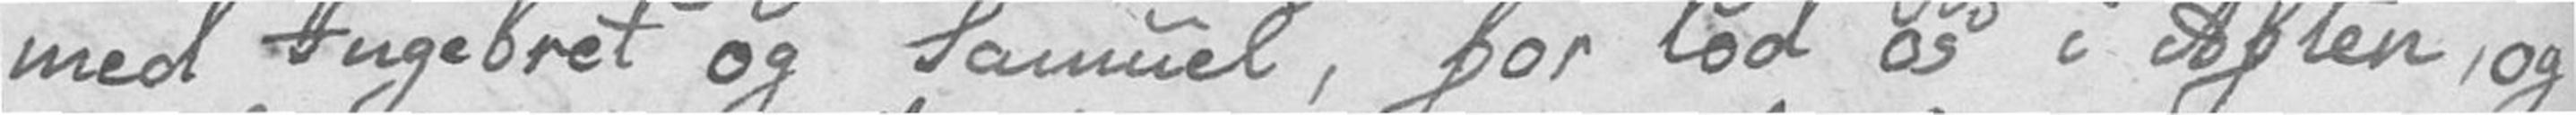med Ingebret og Samuel, for lod os i Aften, og
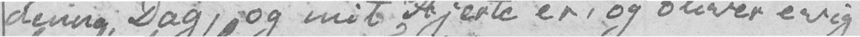denne Dag, og mit Hjerte er, og bliver evig
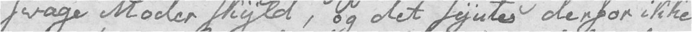svage Moder skyld, og det syntes derfor ikke
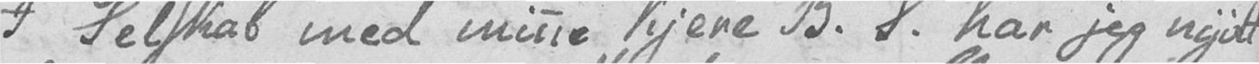I Selskab med mine kjere B. S. har jeg nydt
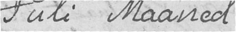Juli Maaned
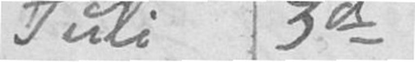Juli 3d
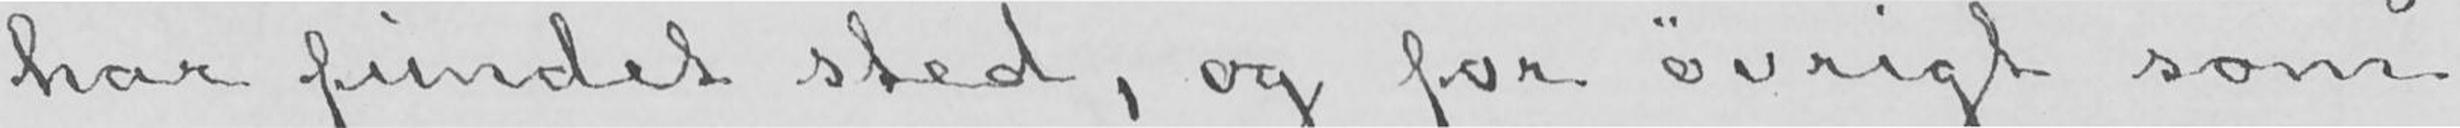har fundet sted, og for øvrigt som
